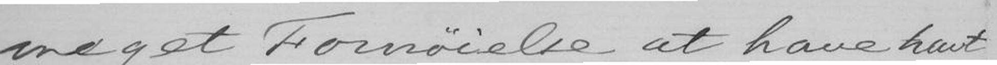meget Fornøielse at have havt
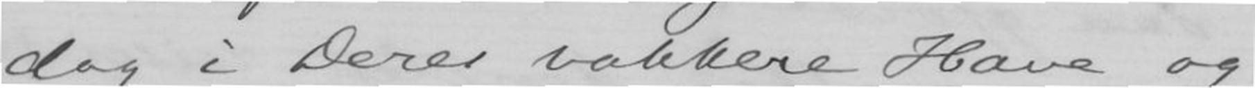dag i Deres vakkre Have og
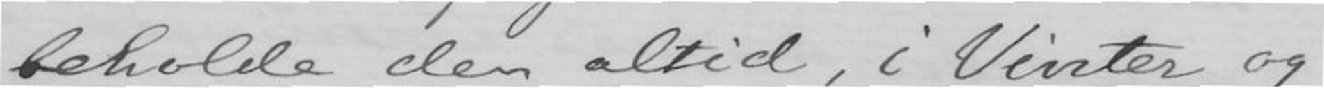beholde den altid, i Vinter og
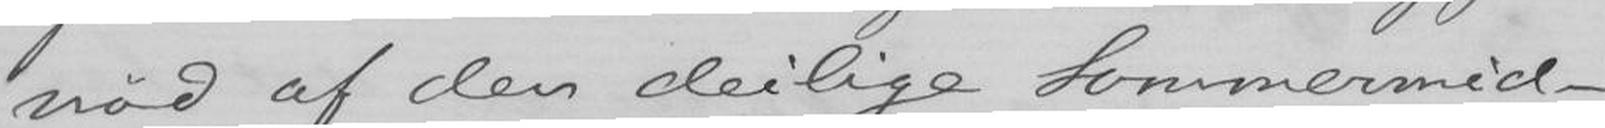nød af den deilige Sommermid-
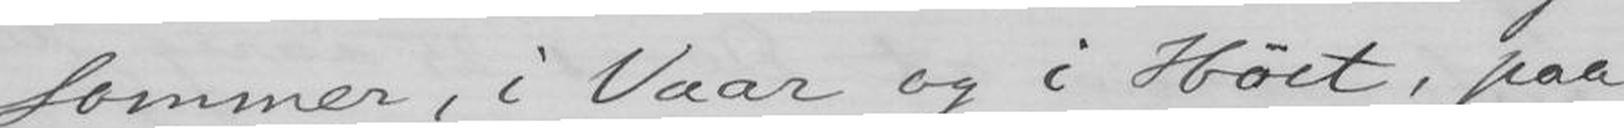Sommer, i Vaar og i Høst, paa
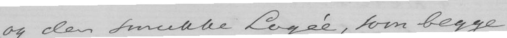og den smukke Logeé, som begge
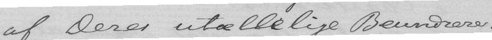af Deres utællelige Beundrere
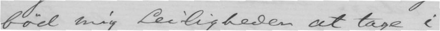bød mig Leiligheden at tage i
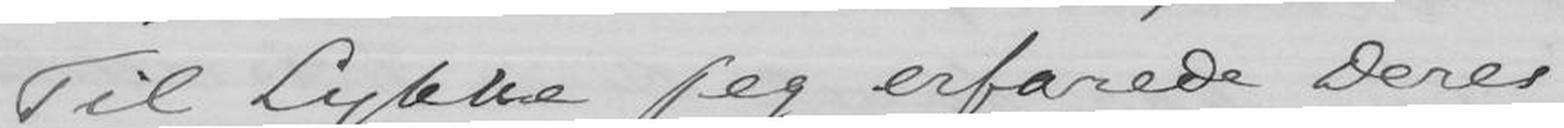Til Lykke jeg erfarede Deres
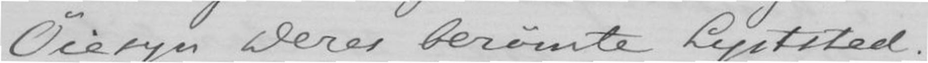Øiesyn Deres berømte Lyststed.
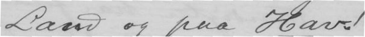Land og paa Hav!
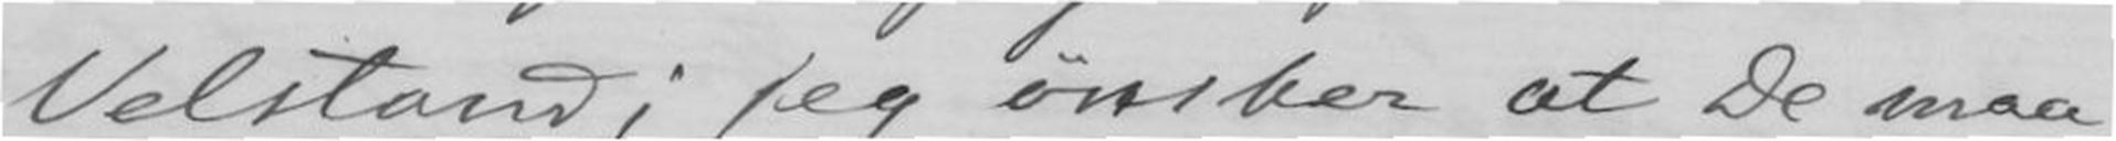Velstand; jeg ønsker at De maa
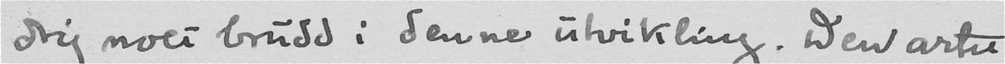drig noet brudd i denne utvikling. Den artet
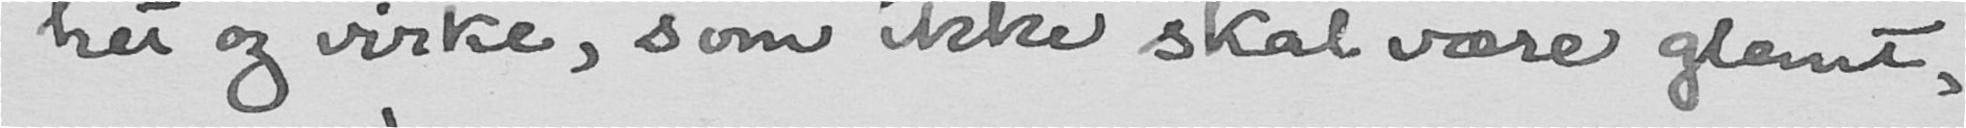het og virke, som ikke skal være glemt,
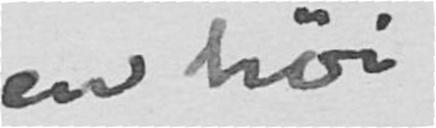en høi
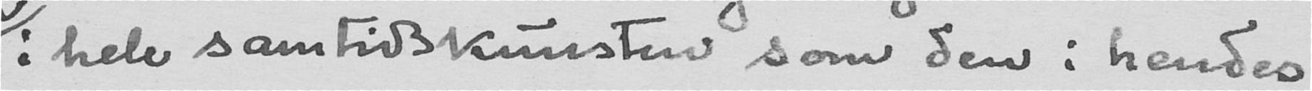i hele samtidskunsten som den i hendes
